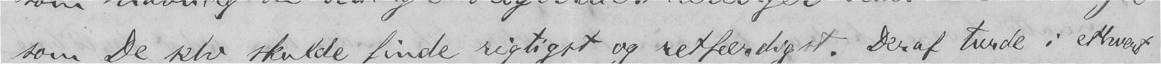som De selv skulde finde rigtigst og retfærdigst. Deraf turde i ethvert
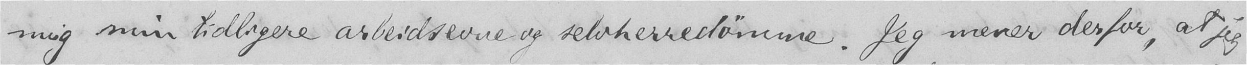mig min tidligere arbeidsevne og selvherredømme. Jeg mener derfor, at jeg
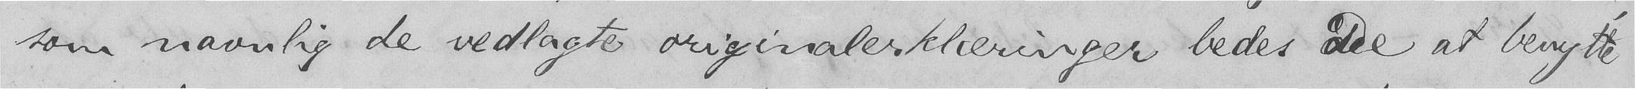som navnlig de vedlagte originalerklæringer bedes De at benytte
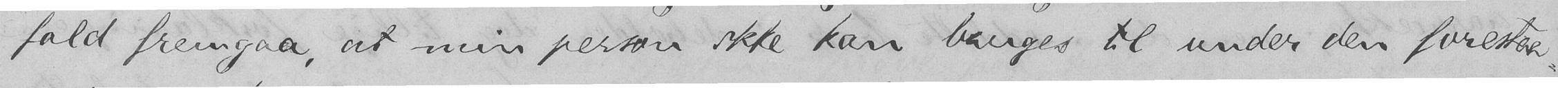fald fremgaa, at min person ikke kan bruges til under den forestaa-
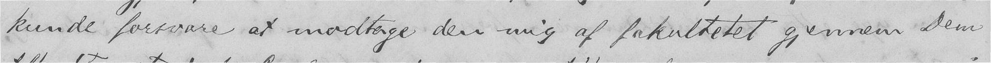kunde forsvare at modtage den mig af fakultetet gjennem Dem
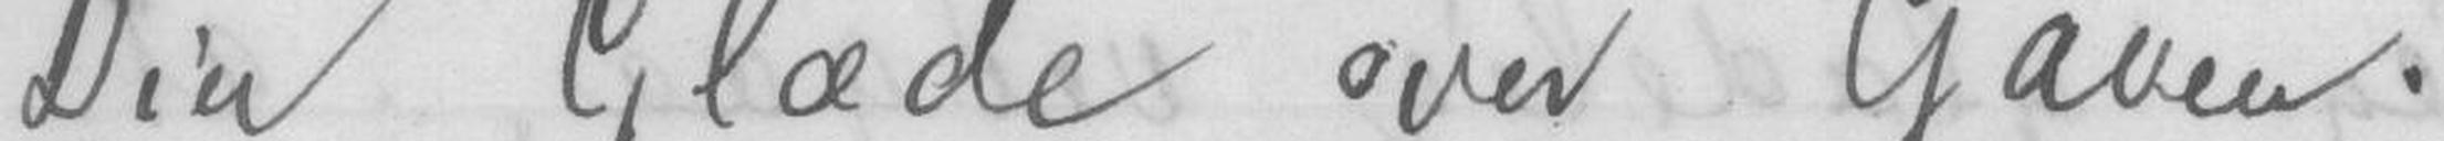Din Glæde over Gaven.
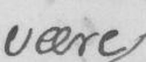være
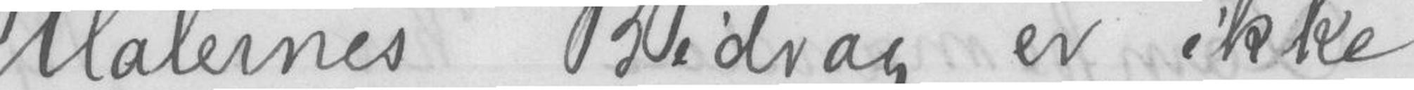Malernes Bidrag er ikke
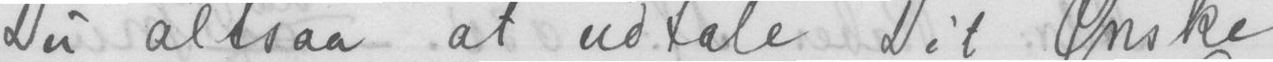Du altsaa at udtale Dit Ønske
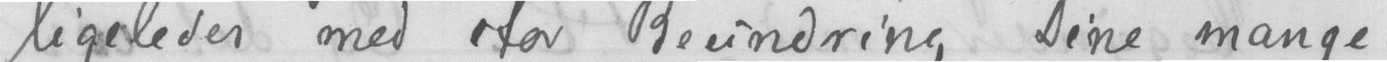ligelædes med stor Beundring Dine Mange
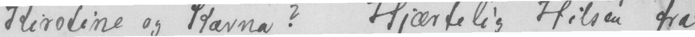Hirodine og Havna? Hjærtelig Hilsen fra
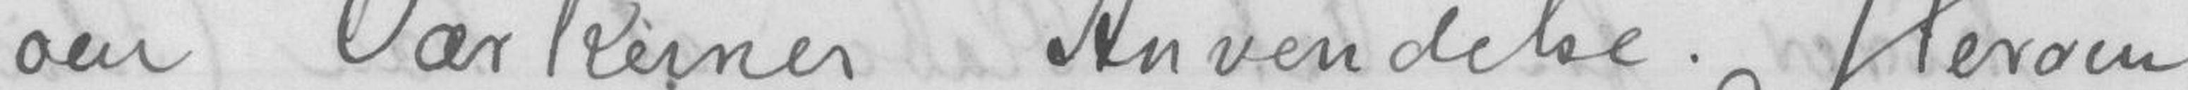om Værkernes Anvendelse. Herover
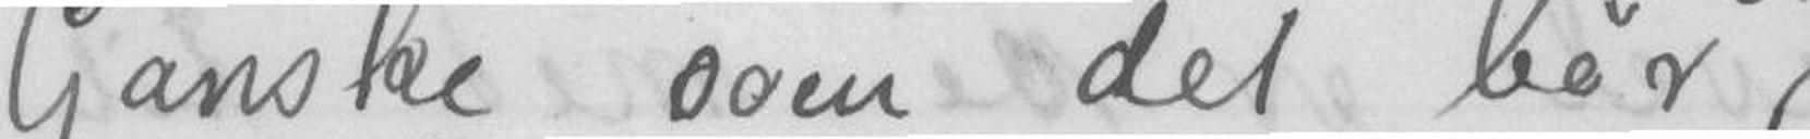Ganske som det bør
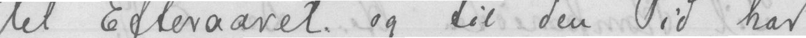til Efteraret og til den Tid har
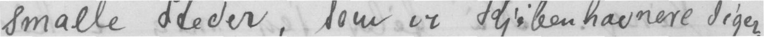smalle Steder, som vi Kjøbenhavnere siger.
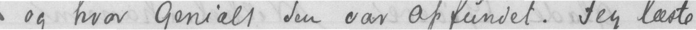og hvor Genialt den var opfundet. Jeg læste
